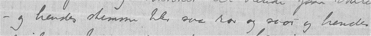- og hendes stemme blev saa rar og saar og hendes
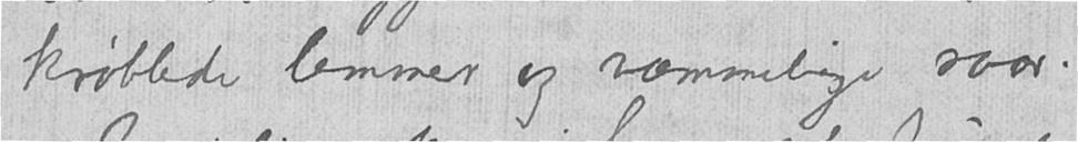krøblede lemmer og væmmelige saar.
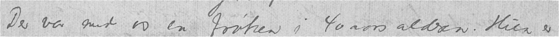Der var med os en frøken i 40aarsalderen. Hun er
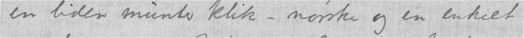en liden munter klik - norske og en enkelt
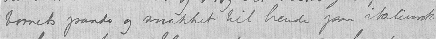barnets pande og snakket til hende paa italiensk
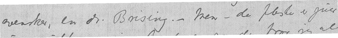svenske, en dr. Brising. - Men - de fleste er pur
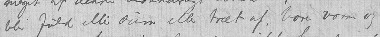blir fuld eller dum eller træt af, bare varm og
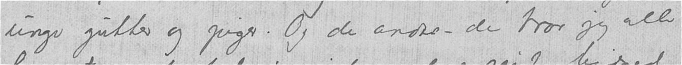unge gutter og piger. Og de andre - de tror jeg alle
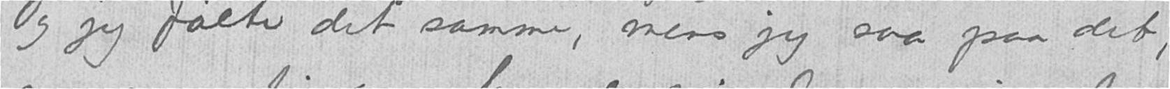Og jeg følte det samme, mens jeg saa paa det,
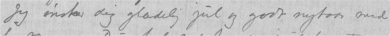Jeg ønsker dig glædelig jul og godt nytaar med
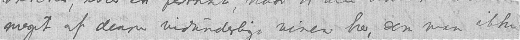meget af denne vidunderlige vinen her, som man ikke
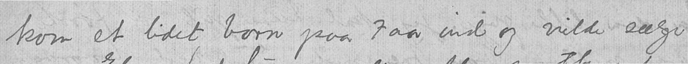kom et lidet barn paa 7 aar ind og vilde sælge
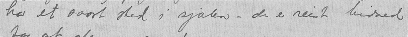har et saart sted i sjælen - de er reist hidned
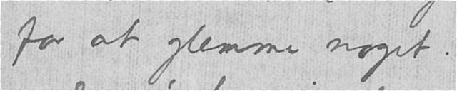for at glemme noget.
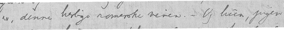er, denne herlige romerske vinen.  - Og hun, pigen
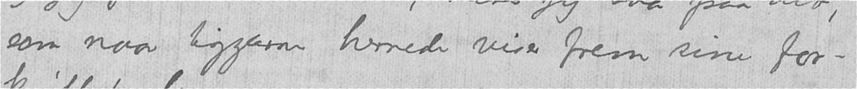som naar tiggerne hernede viser frem sine for-
